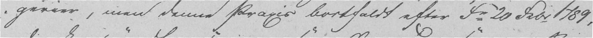gerier, men denne Praxis bortfaldt efter Fr 20 Febr 1789,
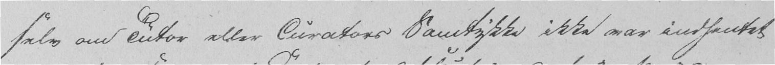selv om Tutor eller Curators Samtykke ikke var indhentet
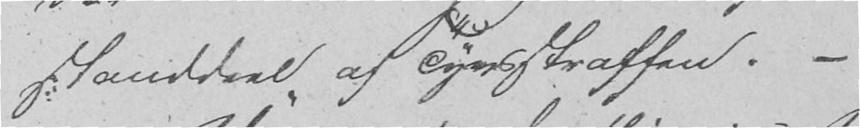standdeel af Tyvstraffen. -
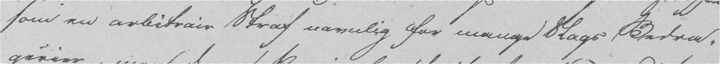som en arbitrair Straf navnlig for mange Slags Bedra-
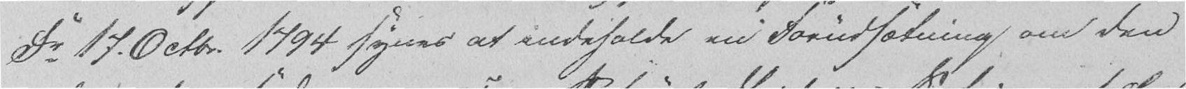Fr 17. Octbr 1794 synes at indeholde en Forudsætning om den
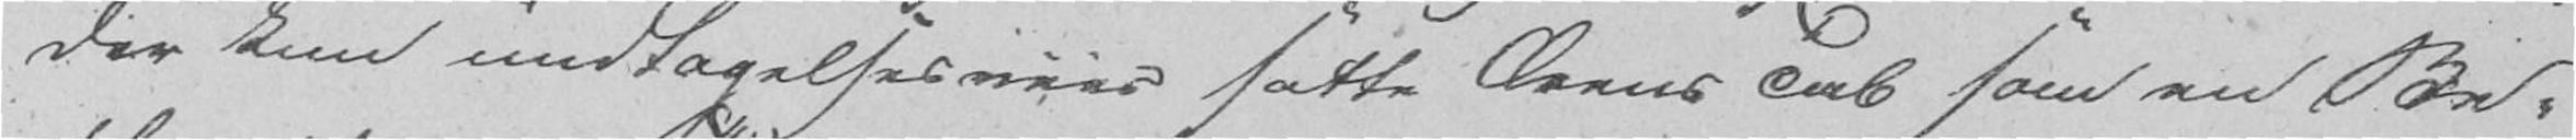der kun undtagelsesviis satte Ærens Tab som en Be-
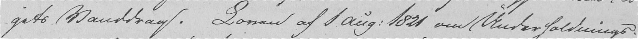gets Vanddrag. Loven af 1 Aug. 1821 om Underholdnings-
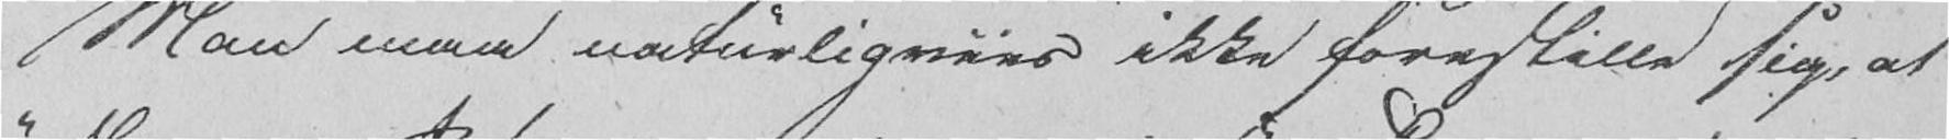Man maa naturligviis ikke forestille sig, at
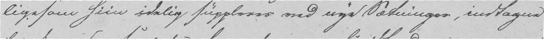ligesom hiin idelig supplerer med nye Sætninger, indtagen
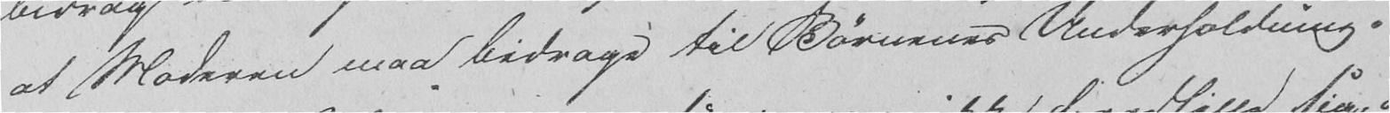at Moderen maa bidrage til Børnenes Underholdning.
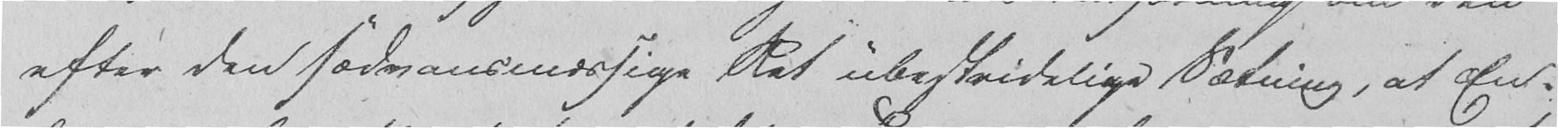efter den sædvansmæssige Ret ubestridelige Sætning, at En-
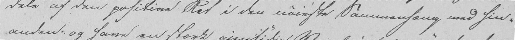dele af den positive Ret i den nøieste Sammenhæng med hin-
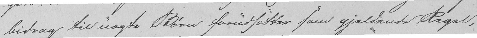bidrag til uægte Børn forudsætter som gjeldende Regel,
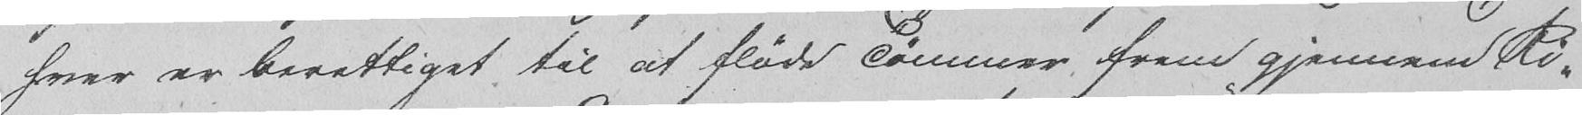hver er berettiget til at fløde Tømmer frem gjennem Ri-
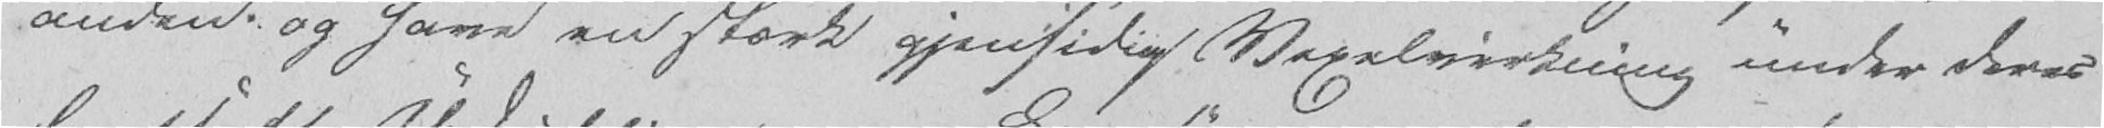anden og have en stærk gjensidig Vexelvirkning under deres
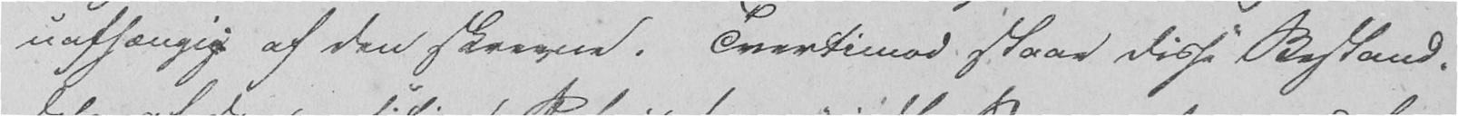uafhængig af den skrevne. Tvertimod staar disse Bestand-
